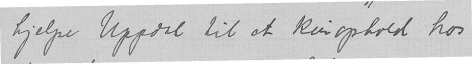hjelpe Uppdal til et kurophold hos
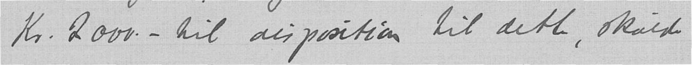Kr. 2000. – til disposition til dette, skulde
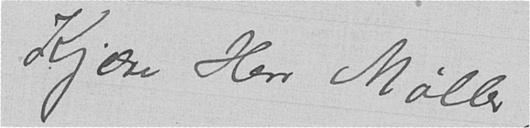Kjære Herr Møller,
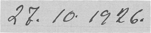27.10.1926.
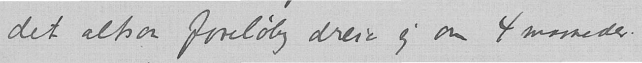det altsaa foreløbig dreie sig om 4 maaneder.
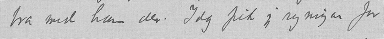bra med ham der. Idag fik jeg regningen for
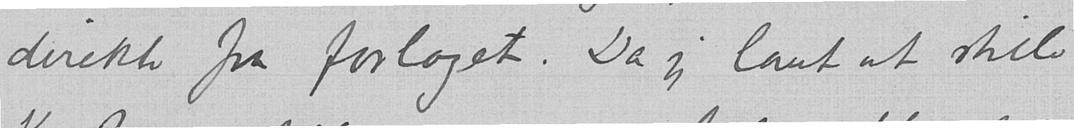direkte fra forlaget. Da jeg lovet at stille
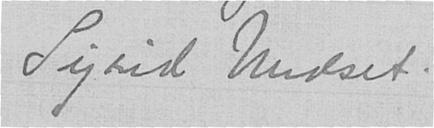Sigrid Undset.
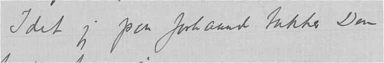Idet jeg paa forhaand takker Dem
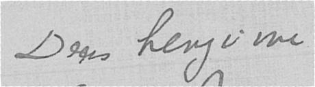Deres hengivne
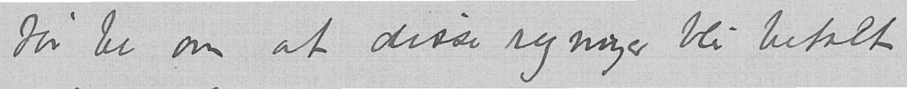tør be om at disse regninger blir betalt
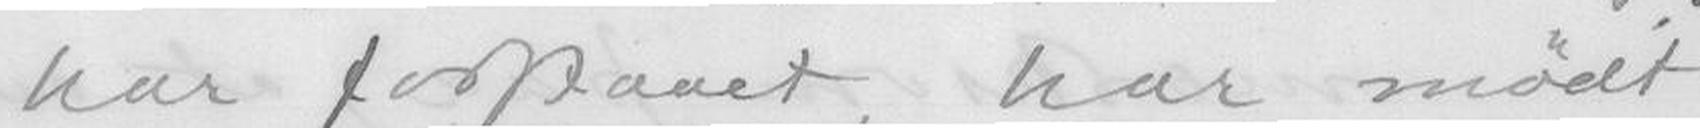har forstaaet, har mødt
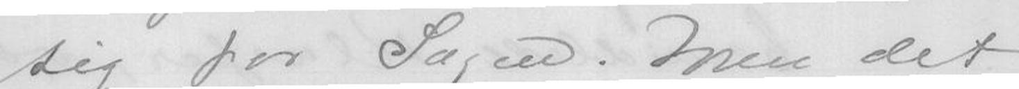sig for Sagen. Men det
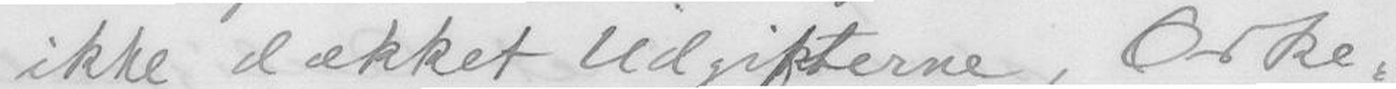ikke dækket Udgifterne, Orke-
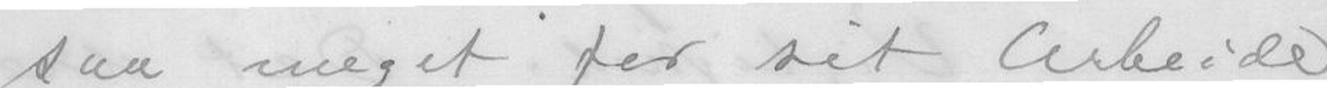saa meget for sit Arbeide,
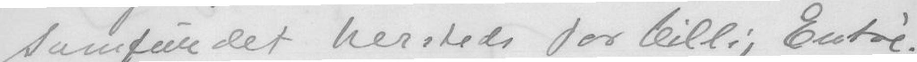samfundet hersteds for billig Entré.
